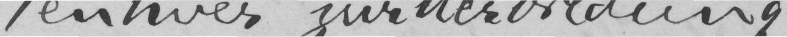enhver zwitterbildung
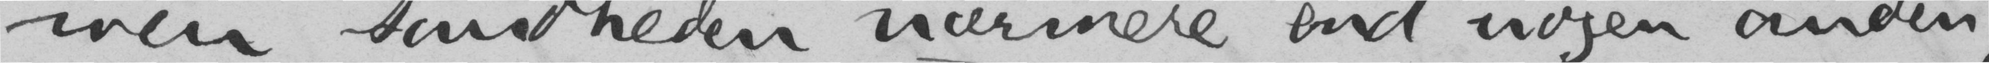men sandheden nærmere end nogen anden,
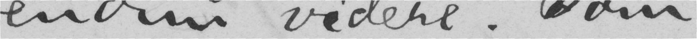endnu videre. Som
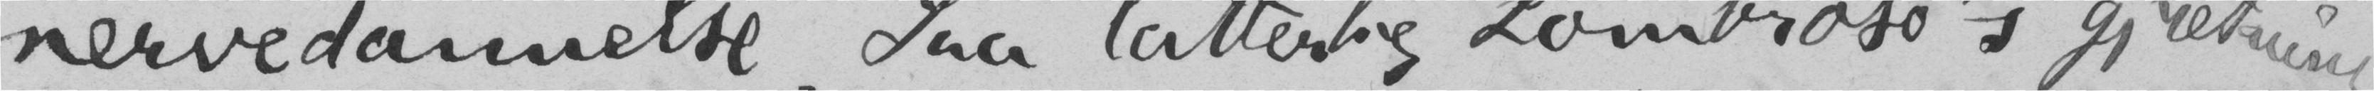nervedannelse. Saa latterlig Lombroros’s gjætning
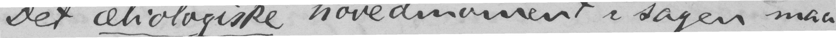Det ætiologiske hovedmoment i sagen maa,
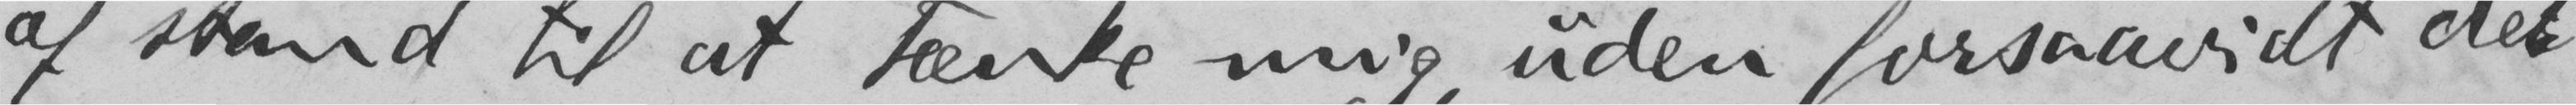af stand til at tænke mig, uden forsaavidt det
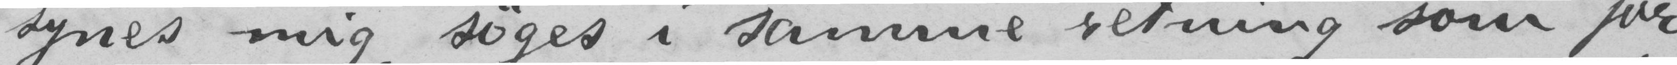synes mig, søges i samme retning som for
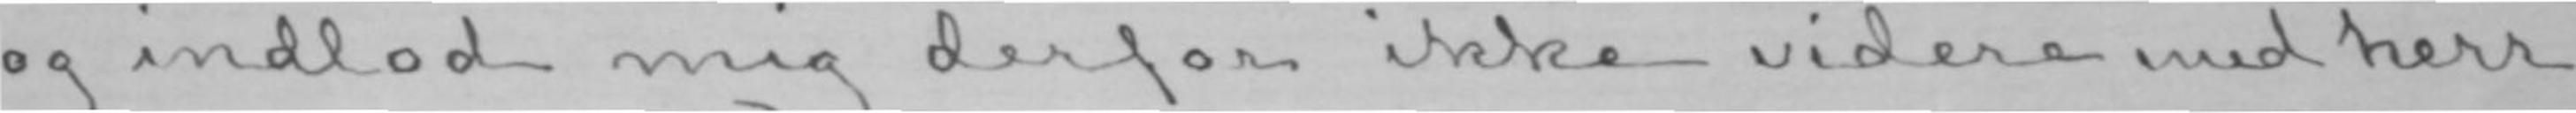og indlod mig derfor ikke videre med herr
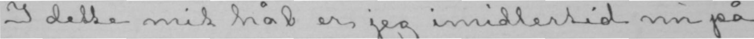I dette mit håb er jeg imidlertid nu på
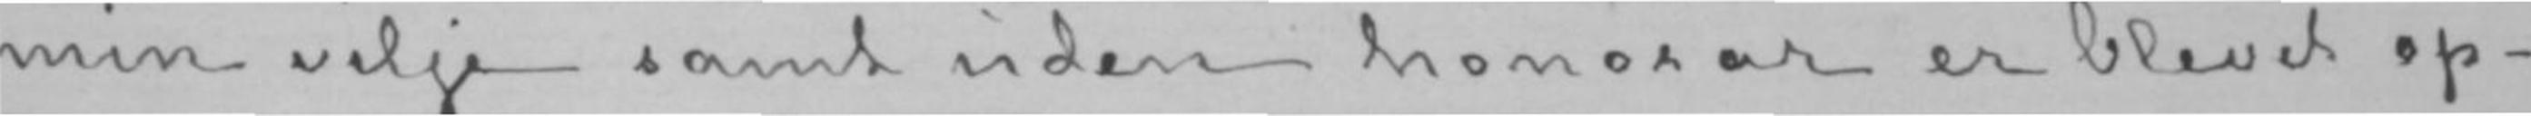min vilje samt uden honorar er blevet op-
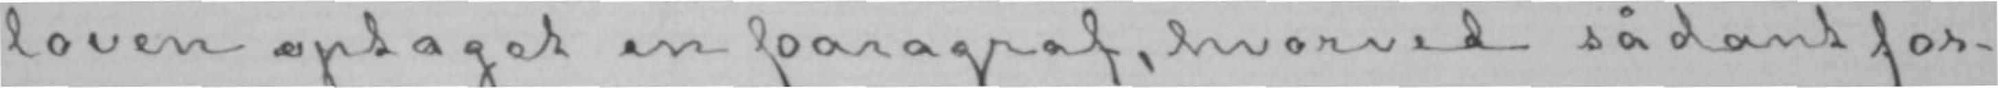loven optaget en paragraf, hvorved sådant for-
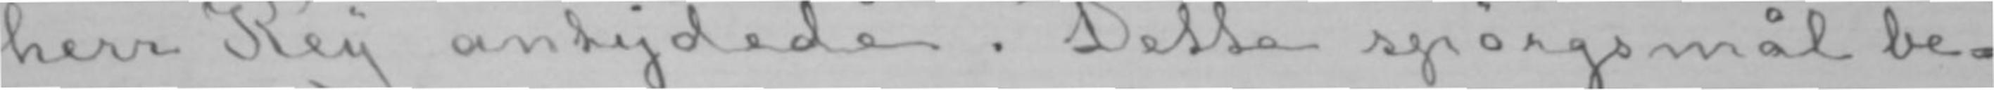herr Key antydede. Dette spørgsmål be-
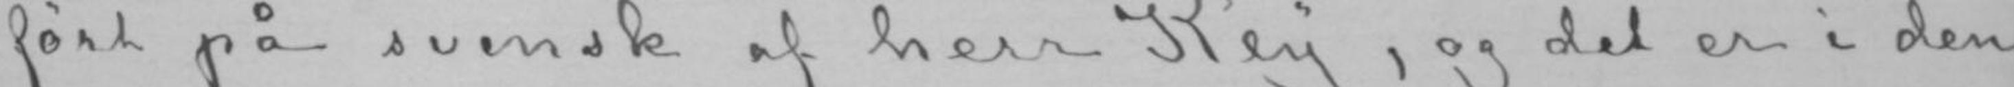ført på svensk af herr Key, og det er i den
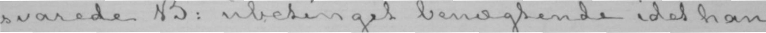svarede B: ubetinget benægtende idet han
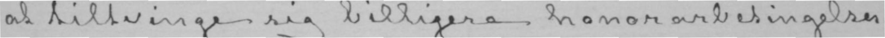at tiltvinge sig billigere honorarbetingelser.
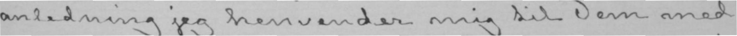anledning jeg henvender mig til Dem med
